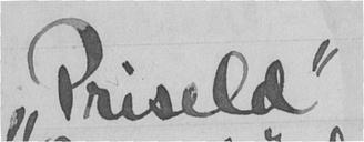"Priseld"
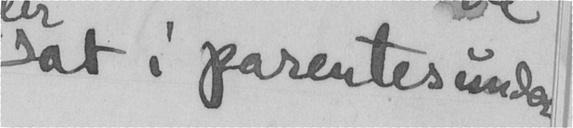sat i parentes under
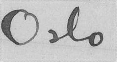Oslo
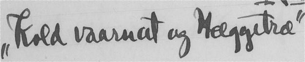"Kold vaarnat og Hæggetræ"
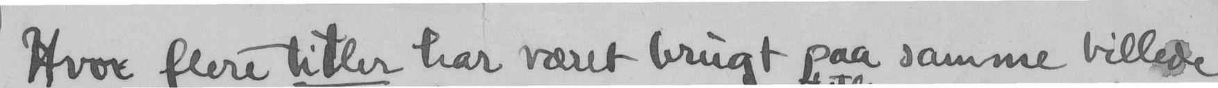Hvor flere titler har været brugt paa samme billede
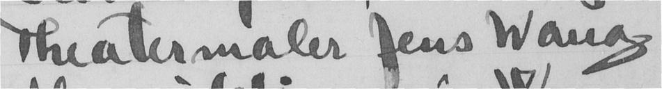Theatemaler Jens Wang
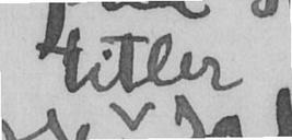titler
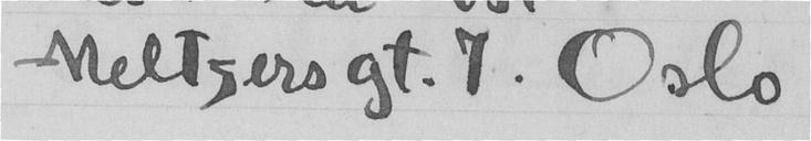Meltzers gt. 7. Oslo
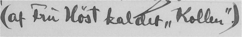(af Fru Høst kaldet "Kollen").
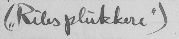("Ribsplukkere")
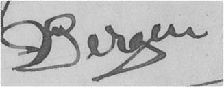Bergen
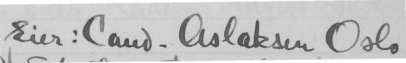Eier: Cand. Aslaksen Oslo
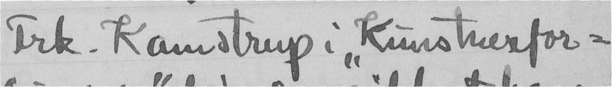Frk. Kamstrup i "Kunstnerfor-
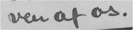ven af os.
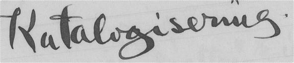Katalogisering.
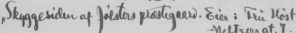"Skyggesiden af Jølsters præstegaard. Eier : Fru Høst
